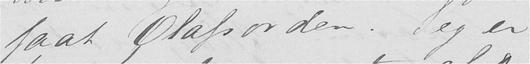faat Olafsorden Jeg er
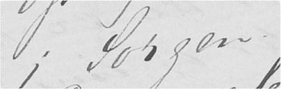i Sorgen.
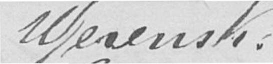Werensk:
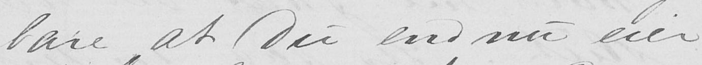bare, at Du endnu eier
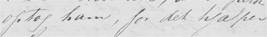optog ham, for det hjælper
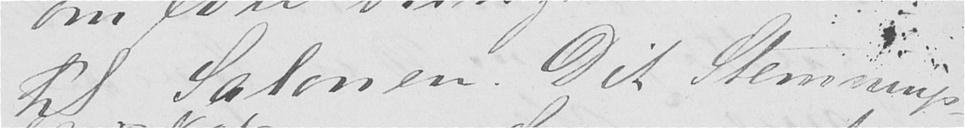til Salonen. Dit Stemnings-
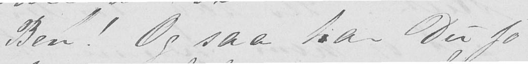Ben! Og saa har Du jo
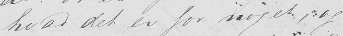hvad det er for noget, og
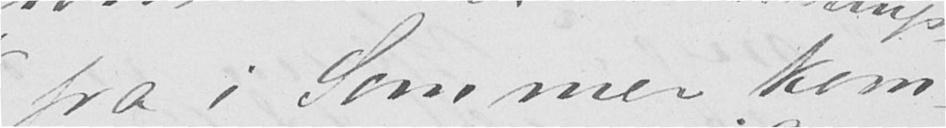fra i Sommer kom-
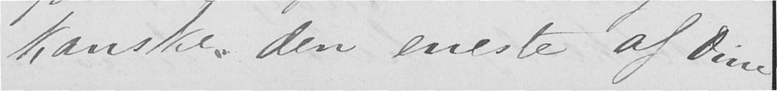kanske den eneste af dine
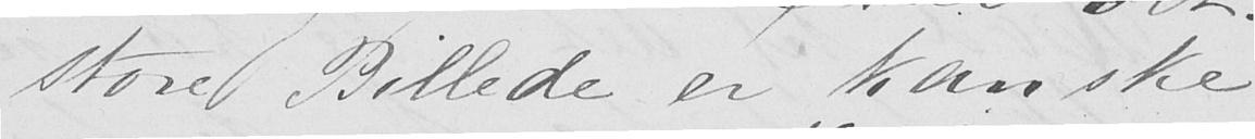store Billede er kanske
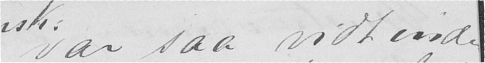var saa vidt inde
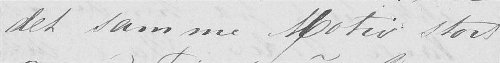det samme Motiv stort
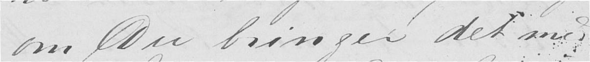om Du bringer det med
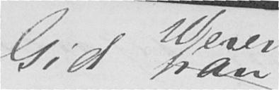Gid han
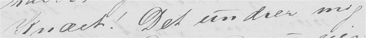Knæet! Det undrer mig
